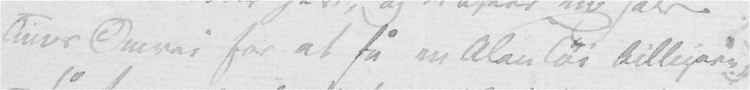Times Omvei for at få en Alen Tøi billigere,
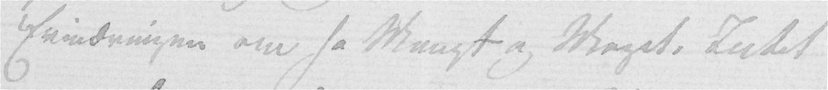Erindringen om sa Mangt og Meget, Intet
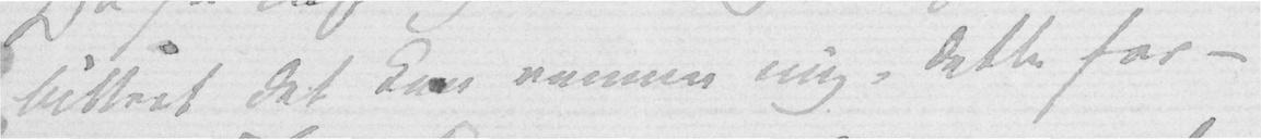bittert det kan ramme mig, dette for-
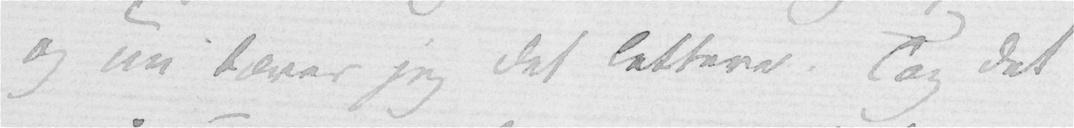og nu bærer jeg det lettere. Tag det
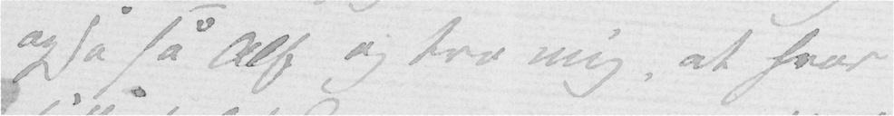også så Alf og tro mig, at hvor
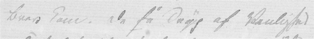Brev kom. De få Dryp af Venlighed
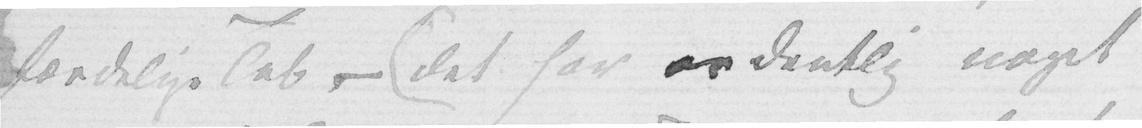færdelige Tab – (det har ordentlig noget
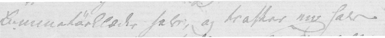Bemmetørklædes selv, og trasker en halv
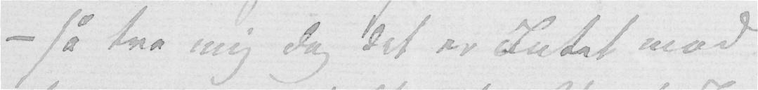– så tro mig dog det er Intet mod
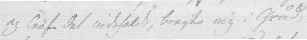og Trøst det indeholdt, bragte mig i Gråd,
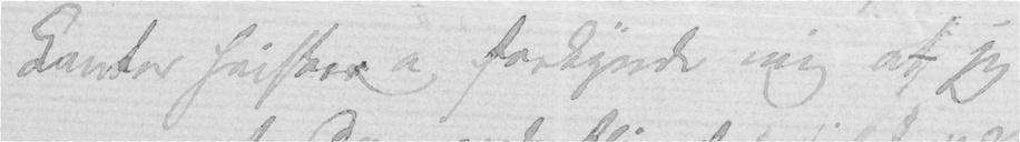Kanter hvisker og forkynder mig at jeg
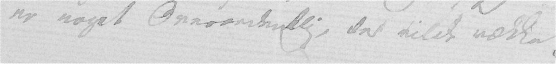er noget Overordentlig, der vilde vækkes
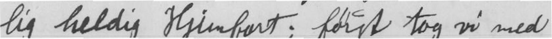lig heldig Hjemfart; først tog vi med
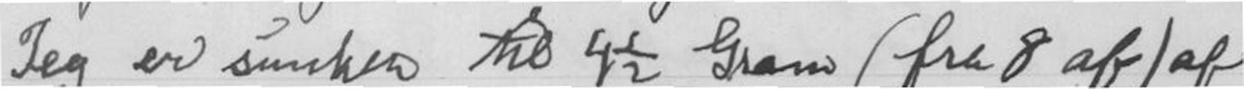Jeg er sunket til 4½ Gram (fra 8 af) af
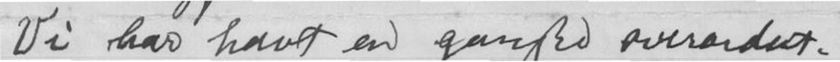Vi har havt en ganske overordent-
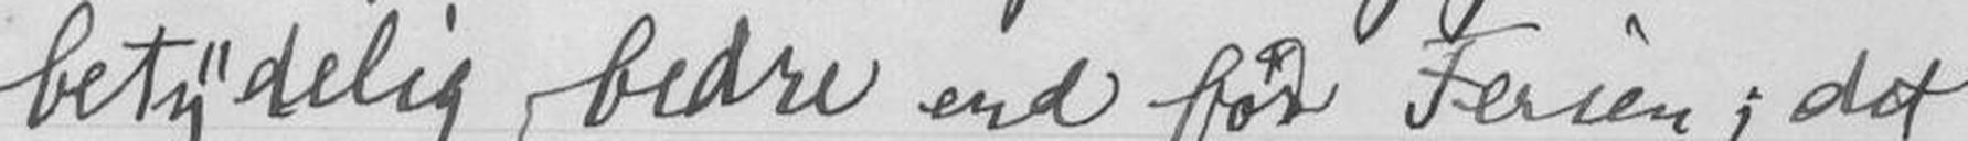betydelig bedre end før Ferien; det
